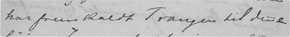har fremkaldt Trangen til denne
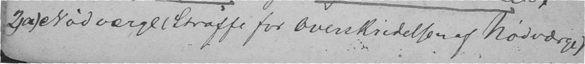2a) Nødværge (Straffe for Overskridelsen af Nødværge)
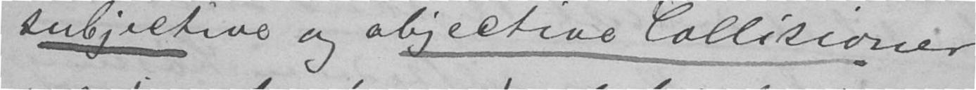subjective og objective Collisioner
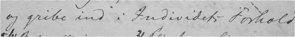og gribe ind i Individets Forhold
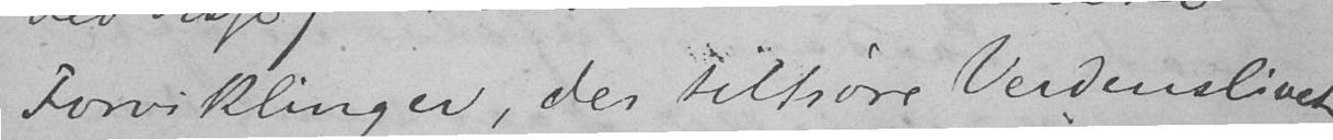Forviklinger, der tilhøre Verdenslivet
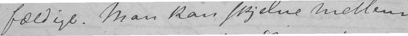fældige. Man kan skjelne mellem
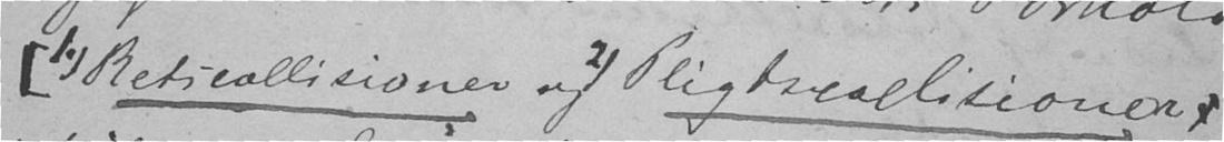[1.) Retscollisioner og 2) Pligtcollitioner
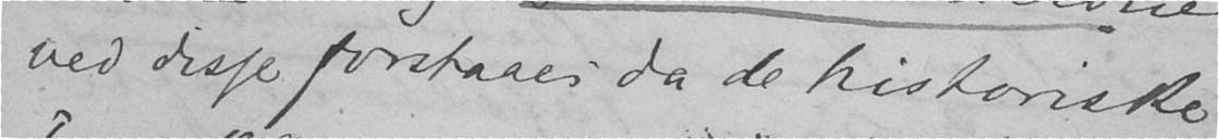ved disse forstaaes da de historiske
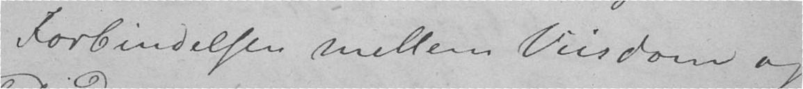Forbindelsen mellem Visdom og
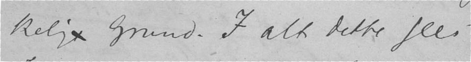kelige Grund. I alt dette sees
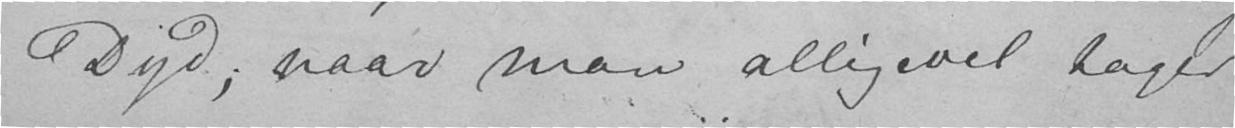Dyd, naar man alligevel tager
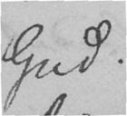Gud. –
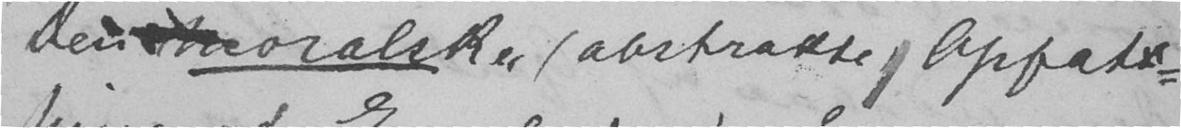Den moralske (abstrakte) Opfat-
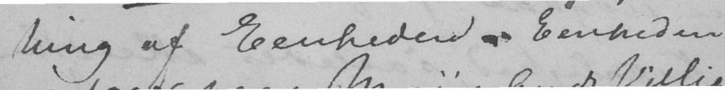ting af Eenheden. Eenheden
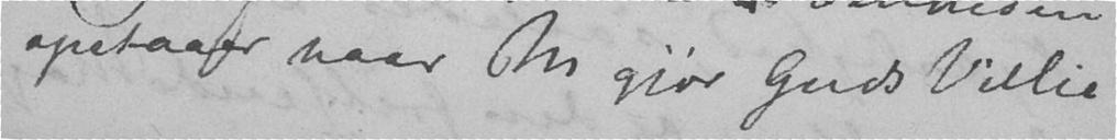opstaaer naar M gjør Guds Villie.
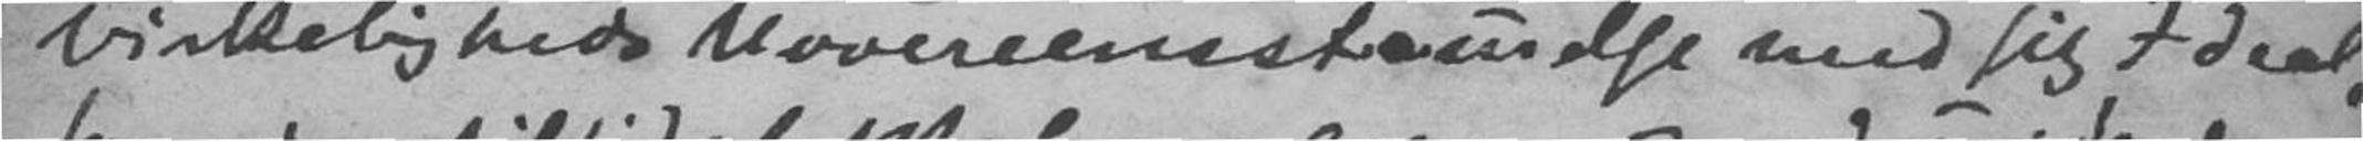Virkeligheds Uovereenssemmelse med sig Ideal,
